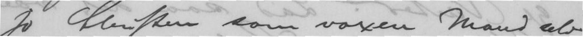jo Christen som voxen Mand selv
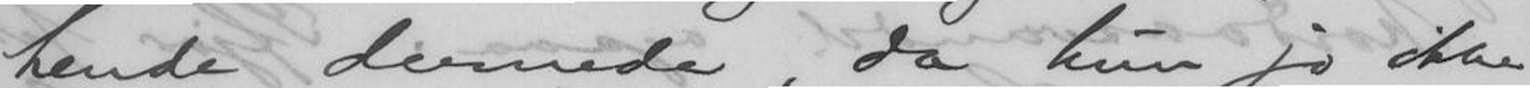hende dernede, da hun jo ikke
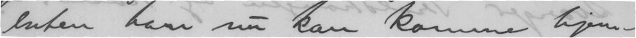enten han nu kan komme hjem-
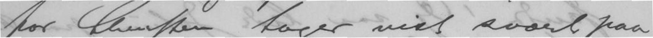for Christen tager vist svært paa
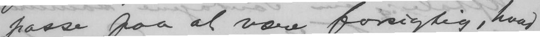passe paa at være forsigtig, hvad
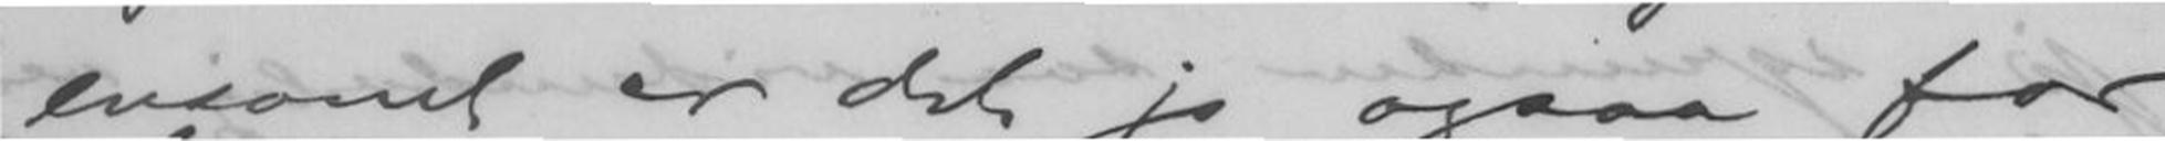ensomt er det jo ogsaa for
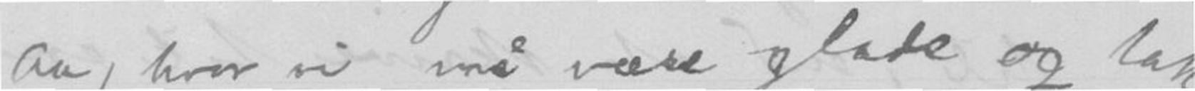Aa, hvor vi må være glade og tak
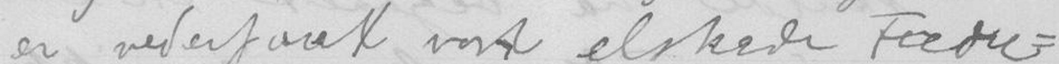er vederfaret vort elskede Fædre-
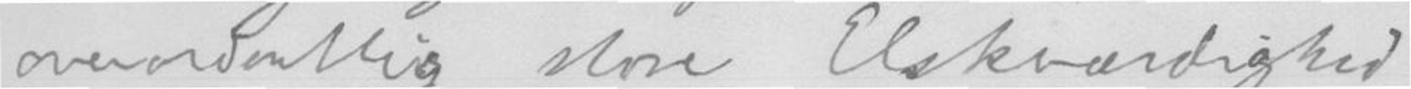overordentlig store Elskværdighed
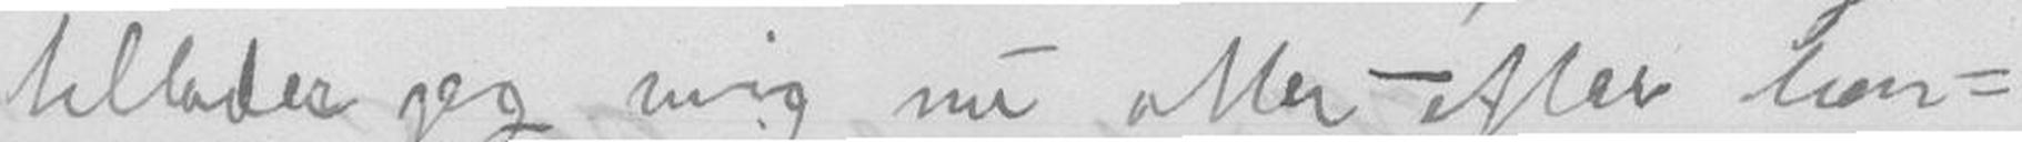tillader jeg mig nu atter - efter læn-
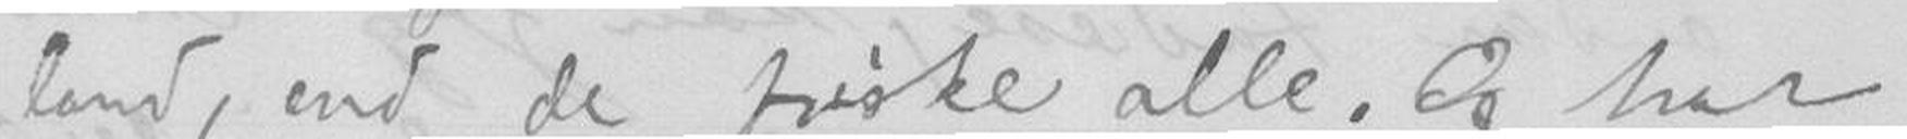land, end de friske alle. Og har
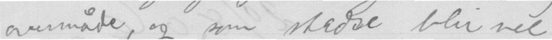overmåde, og som stadse blir vel
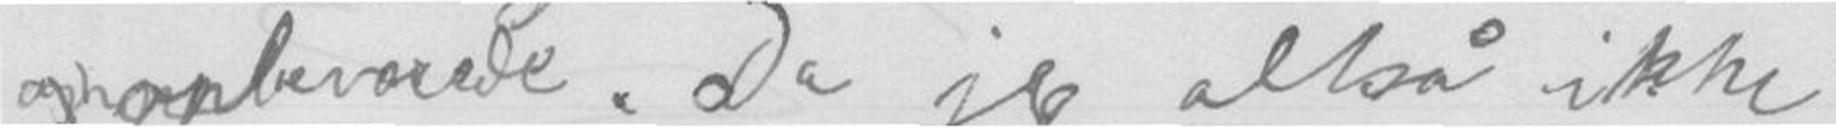op opbevarede. Da jeg altså ikke
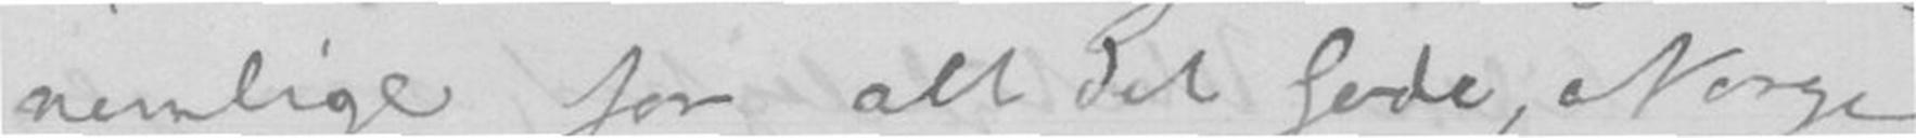nemlige for att det Gode, Norge
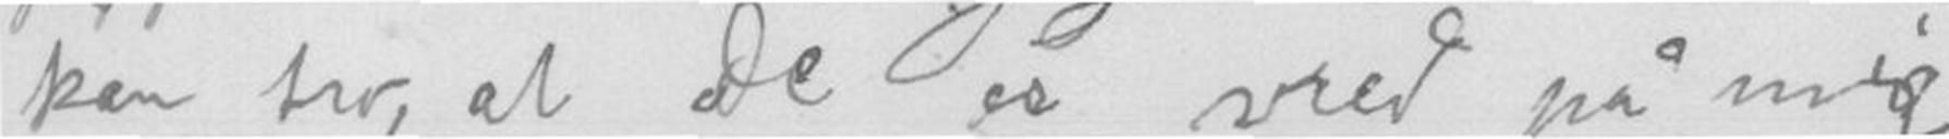kan tro, at De er vred på mig
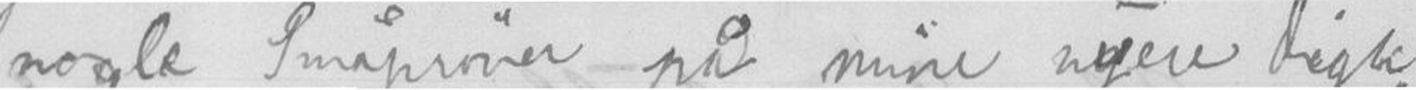nogle Småprøver på mine nyere Digte,
